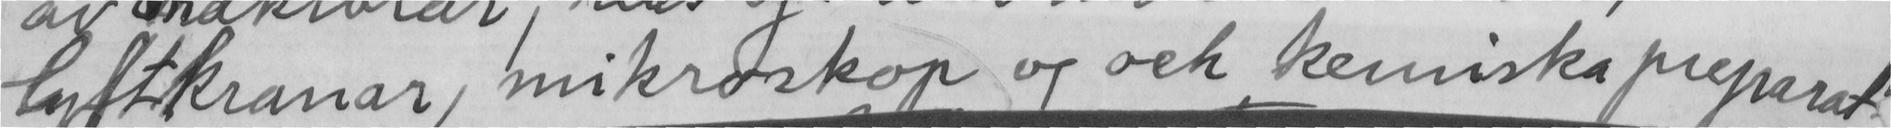lyftkranar, mikroskop og och kemiska preparat."
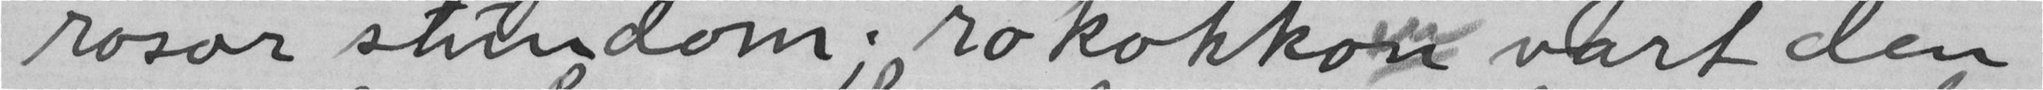rosor stundom. rokokkon vart den
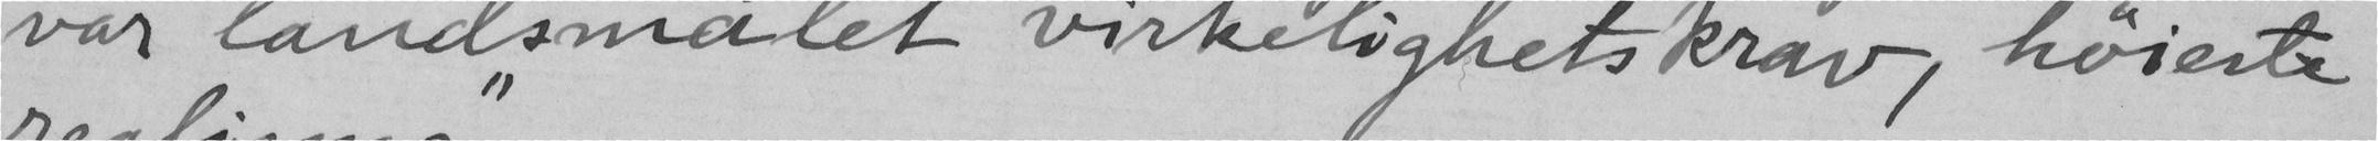var landsmålet virkelighetskrav, høieste
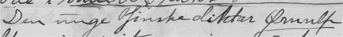- Den unge finske diktar Ørnulf
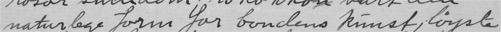naturlege form for bondens kunst, løyste
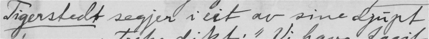Tigerstedt segjer i eit av sine djupt
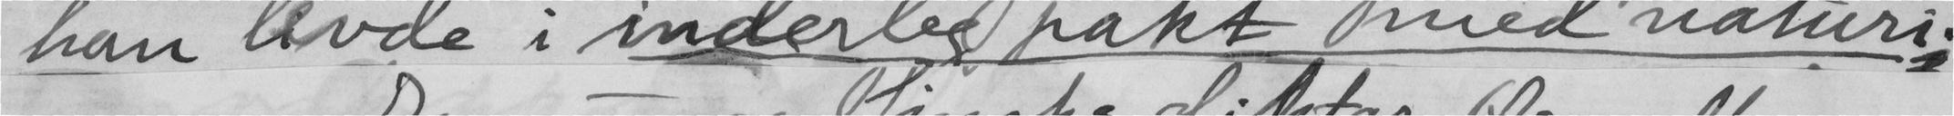han levde i inderleg pakt med naturi.
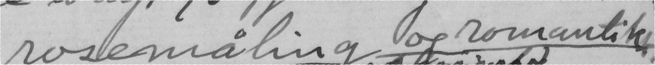rosemåling og romantikk.
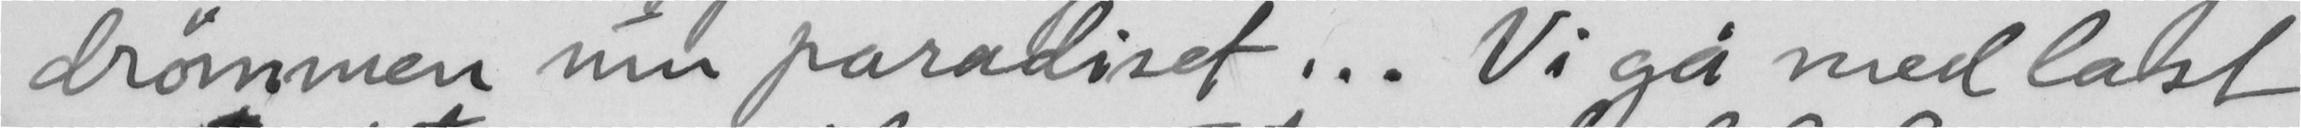drømmen um paradiset... Vi gå med last
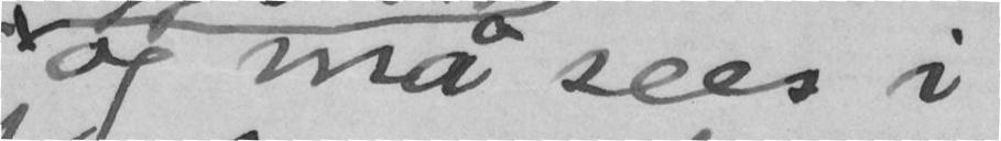og må sees i
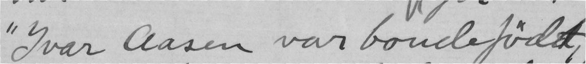"Ivar Aasen var bondefødt,
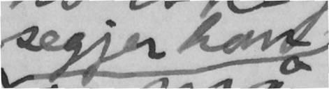segjer han
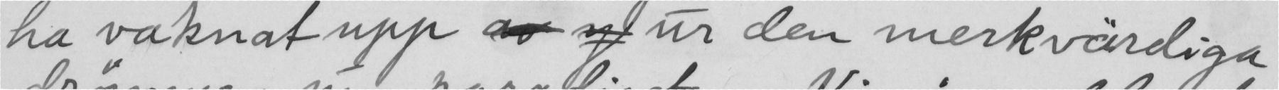ha vaknat upp av og ur den merkvärdiga
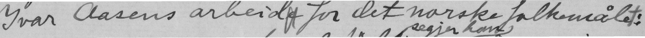Ivar Aasens arbeide for det norske folkemålet:
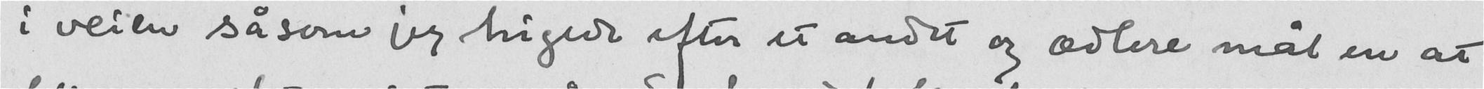i veien såsom jeg higede efter et andet og ædlere mål en at
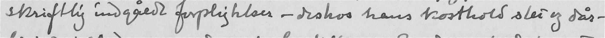skriftlig indgåede forpligtelser - deshos hans kosthold slet og dår-
